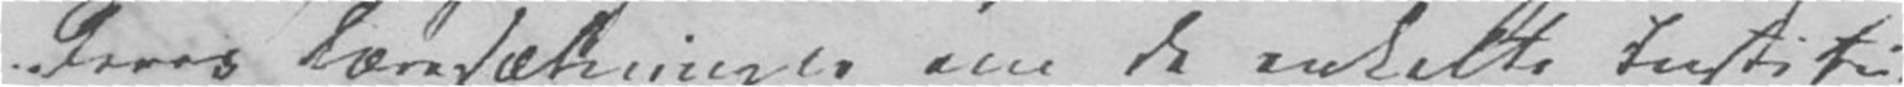deres Læresætninger om de enkelte Institu-
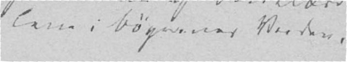leve i Bøgernes Verden,
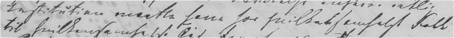Institution maatte have for hvilketsomhelst Folk
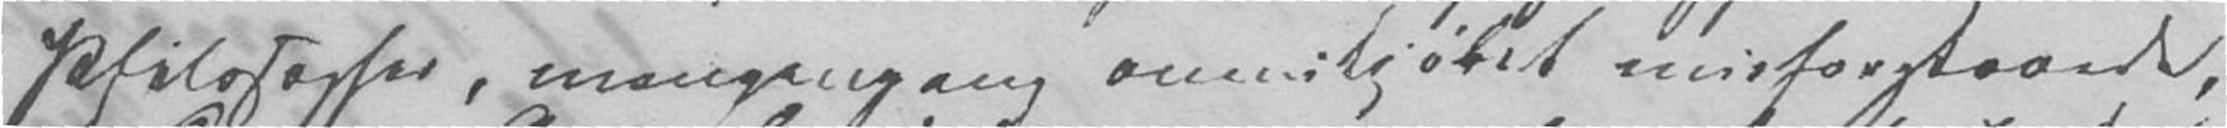Philosopher, mangengang ovenikjøbet misforstaaede,
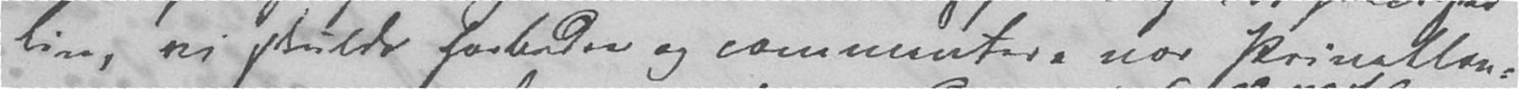Liv, vi skulde forbedre og commentere vor Privatlov-
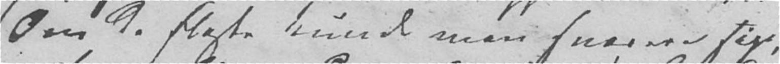Om de fleste kunde man snarere sige,
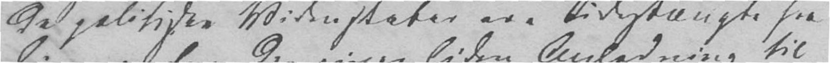de politiske Videnskaber ere udestængte fra
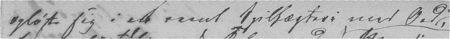opløste sig i et rendt Spilfægteri med Ord,
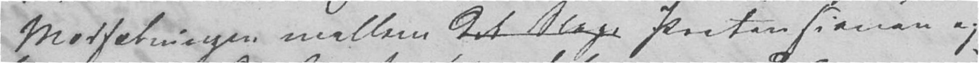Modsætningen mellem Prætensionen og
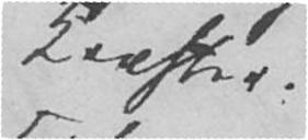Kræfter.
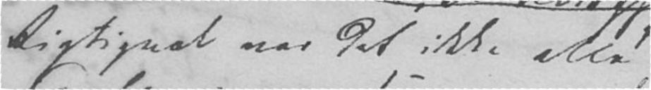Rigtignok var det ikke alle
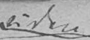uden
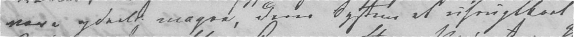vare yderst magre, deres System et ufrugtbart
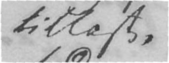tillast.
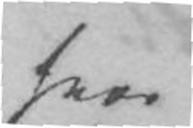hvor
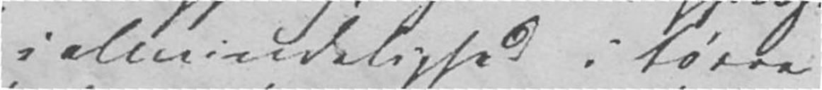i Almindelighed i tørre
